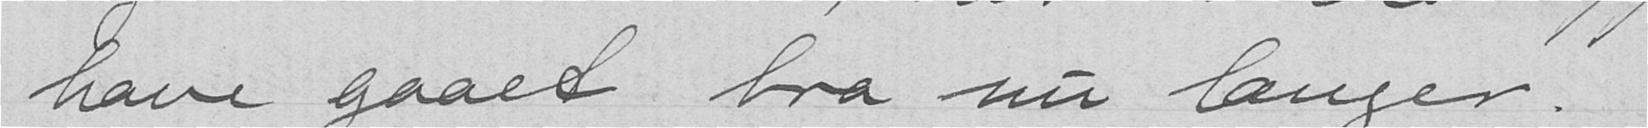have gaaet bra nu længer.
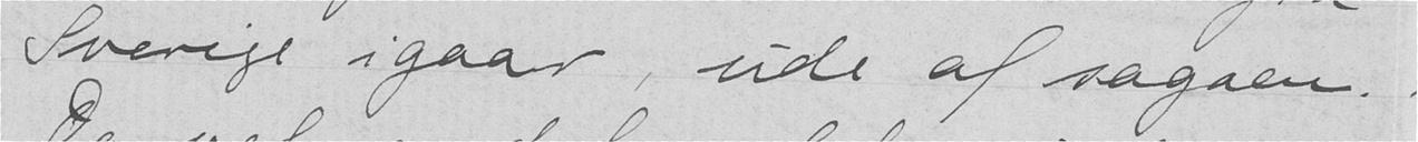Sverige igaar, ude af sagaen.
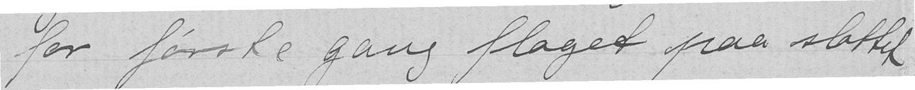for første gang flaget paa slottet
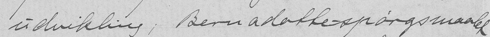udvikling; Bernadotte-spørgsmaalet
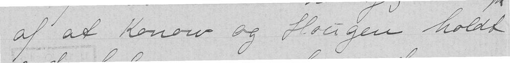af at Konow og Hougen holdt
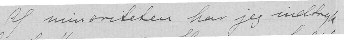Af minoriteten har jeg indtryk
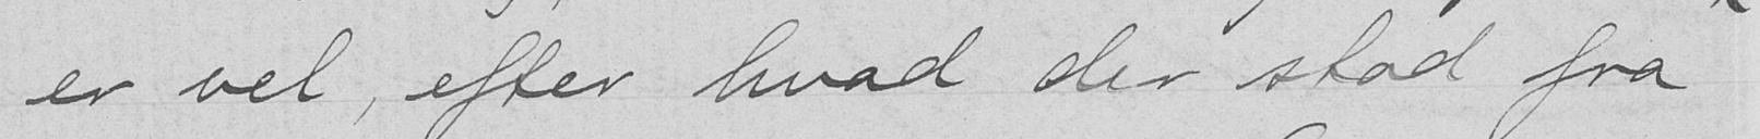er vel, efter hvad der stod fra
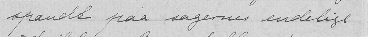spændt paa sagernes endelige
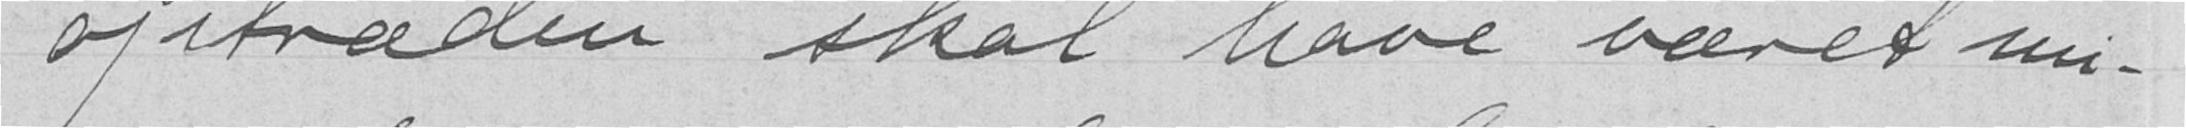optræden skal have været mi-
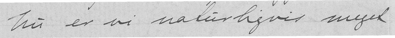- Nu er vi naturligvis meget
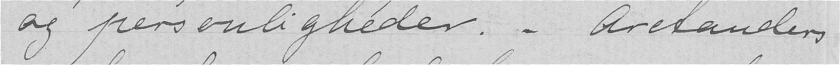og personligheder. - Arctanders
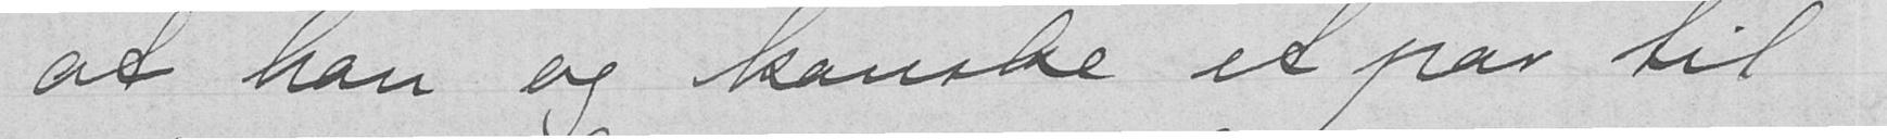at han og kanske et par til
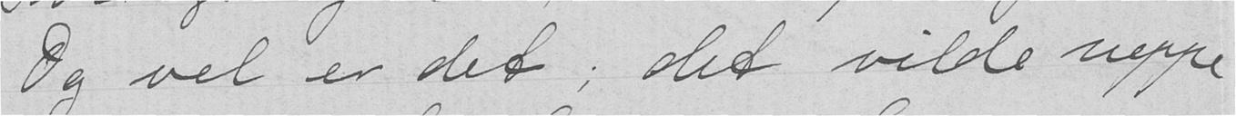Og vel er det; det vilde neppe
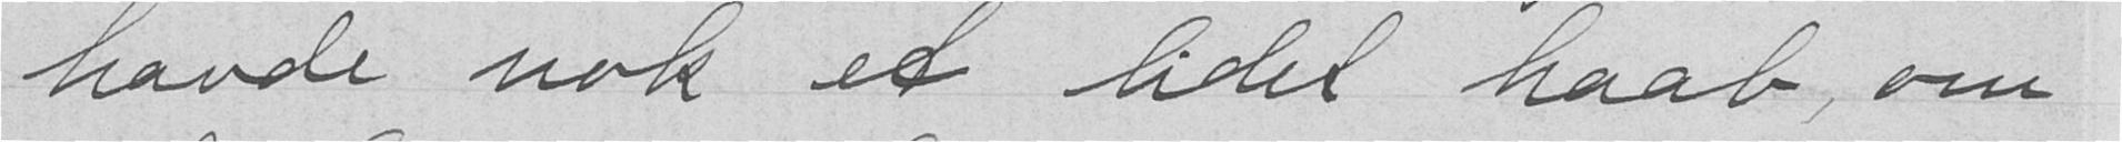havde nok et lidet haab, om
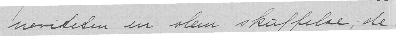noriteten en slem skuffelse; de
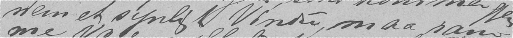nem et synligt Vindu, maa ram-
